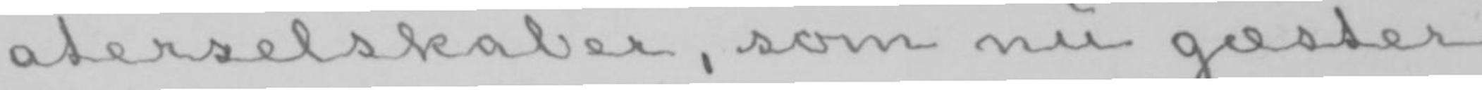aterselskaber, som nu gæster
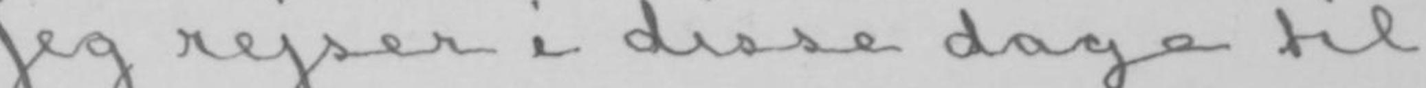Jeg rejser i disse dage til
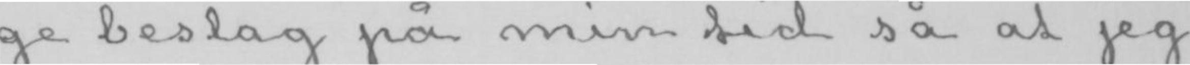ge beslag på min tid så at jeg
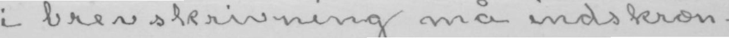i brevskrivning må indskræn-
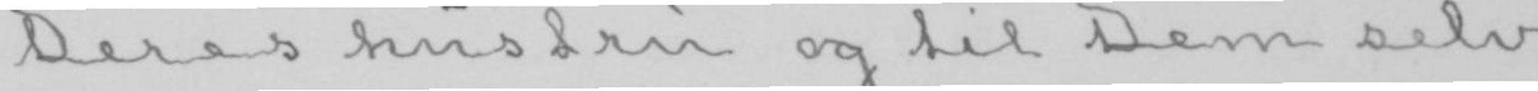Deres hustru og til Dem selv
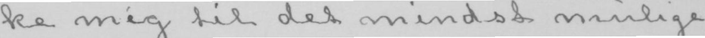ke mig til det mindst mulige.
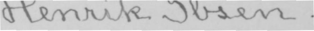Henrik Ibsen.
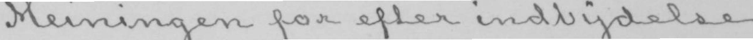Meiningen for efter indbydelse
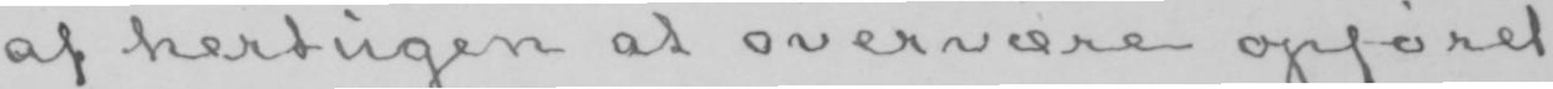af hertugen at overvære opførel-
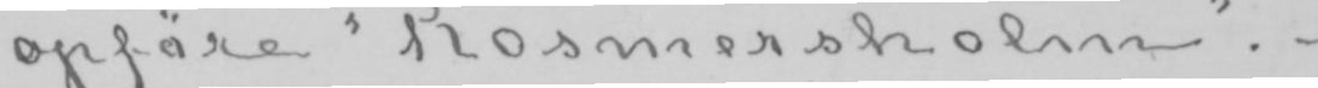opføre «Rosmersholm».-
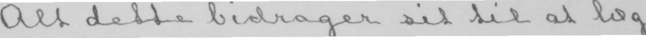Alt dette bidrager sit til at læg-
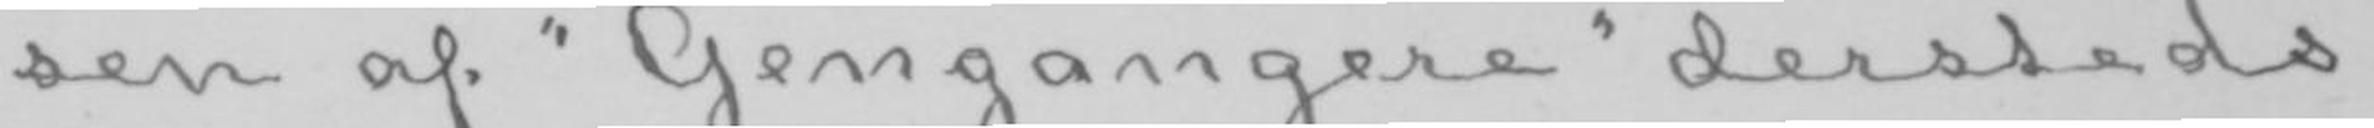sen af «Gengangere» dersteds.
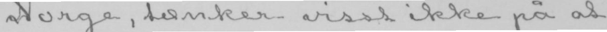Norge, tænker visst ikke på at
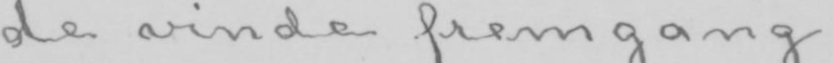de vinde fremgang.
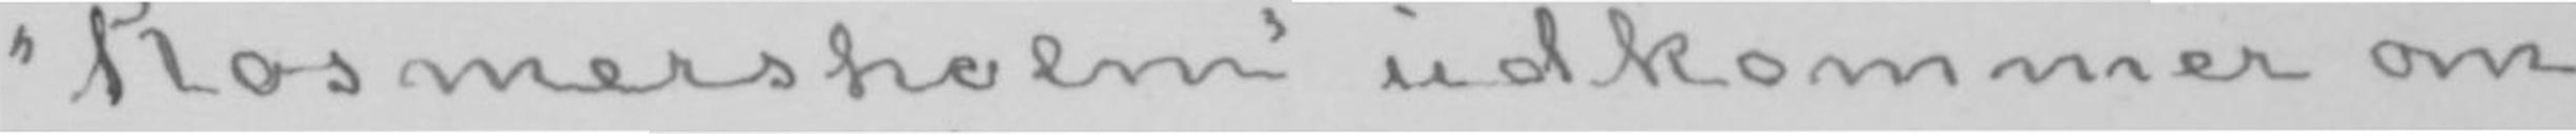«Rosmersholm» udkommer om
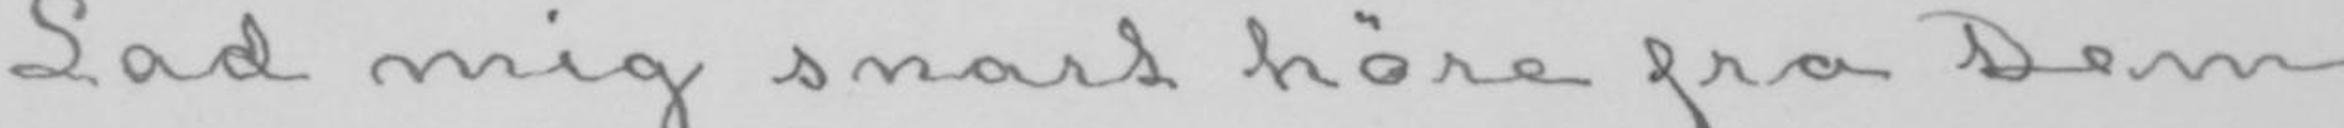Lad mig snart høre fra Dem
